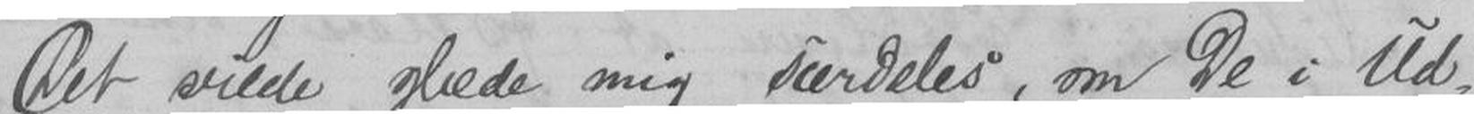Det vilde glæde mig særdeles, og De i Ud-
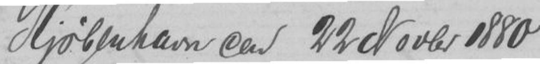Kjøbenhavn Cad 22 Novbr 1880
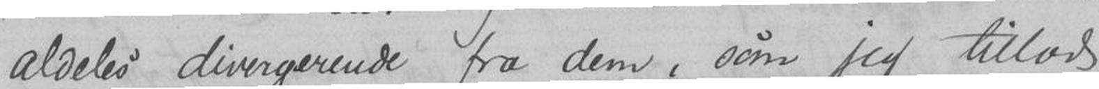aldeles divergerende fra dem, som jeg tillods
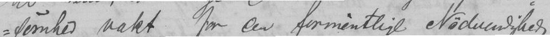somhed vakt for en formintlige nødvendighed
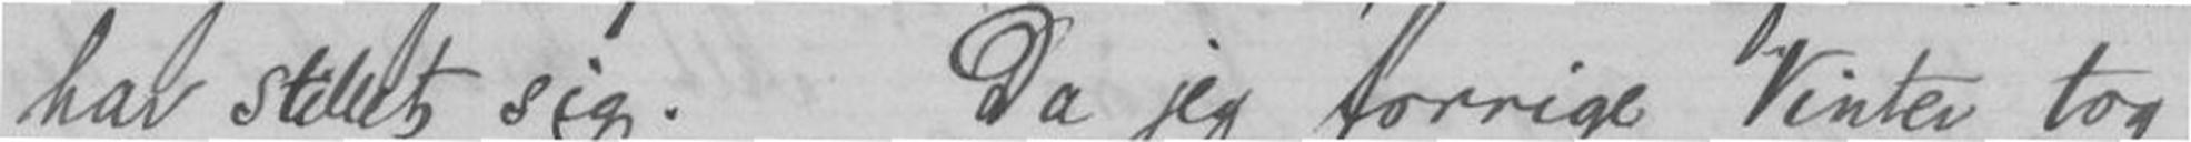har stillet sig.Da jeg forrige Vinter to
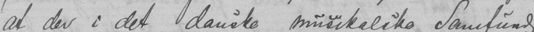at der i det danske musikalske Samfunds
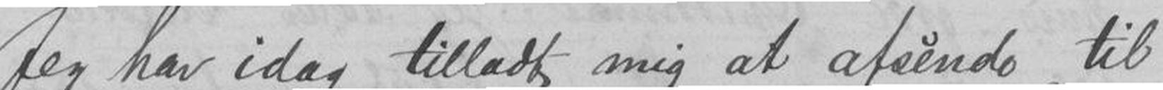Jeg har idag tilladt mig at afsende til
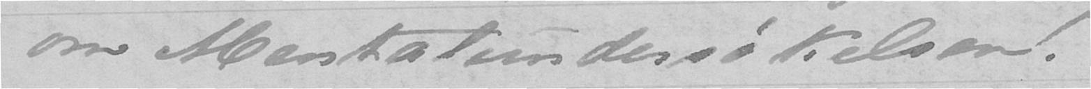om Mentalundersøkelsen!
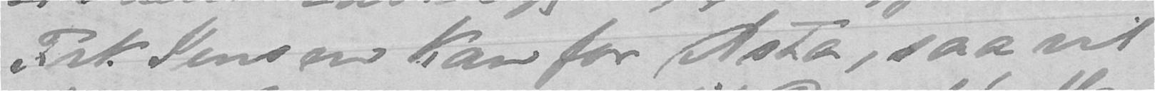Frk Jensen kan for Asta, saa vil
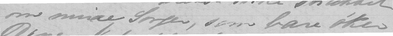om mine Sorger, som bare øker
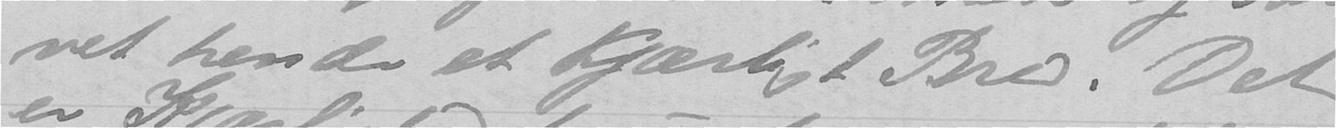vet hende et Kjærligt Brev. Det
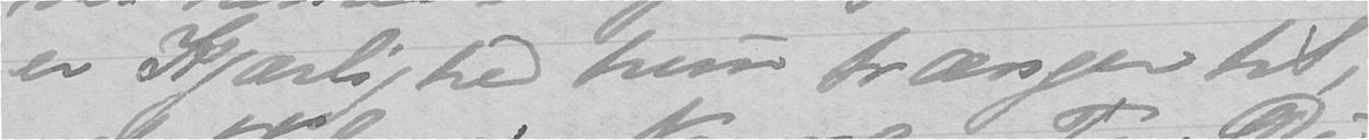er Kjærlighed hun trænger til,
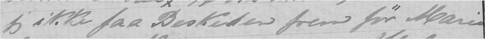jeg ikke faa Beskeden frem før Marie
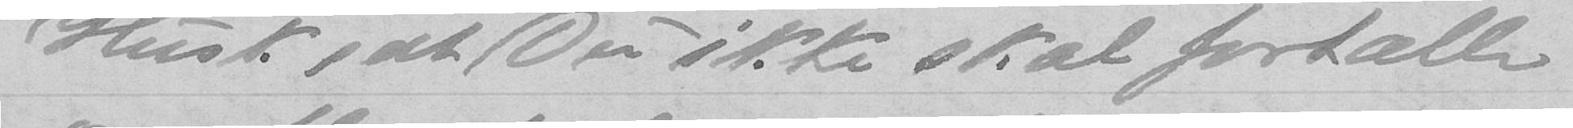Husk, at Du ikke skal fortælle
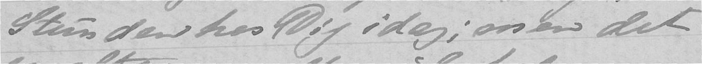Stunden hos Dig idag; men det
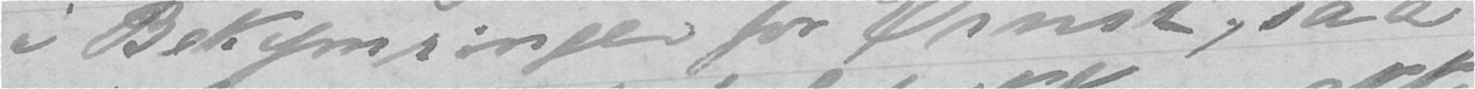i Bekymringer for Ernst, saa
